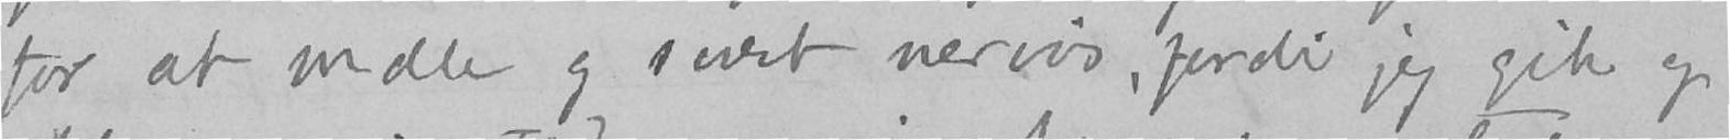for at male og svært nervøs, fordi jeg gik og
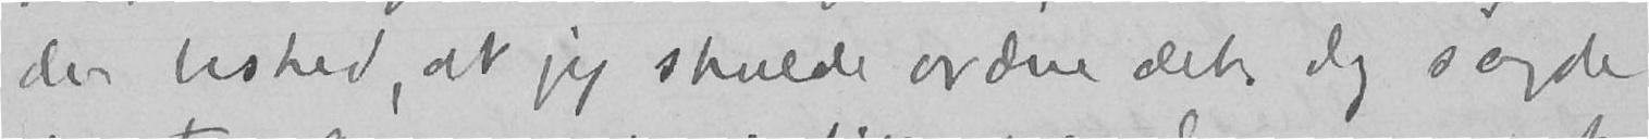den besked, at jeg skulde ordne det jeg sagde
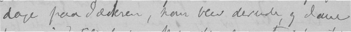dage paa Jæderen, han blev derude, og Jane
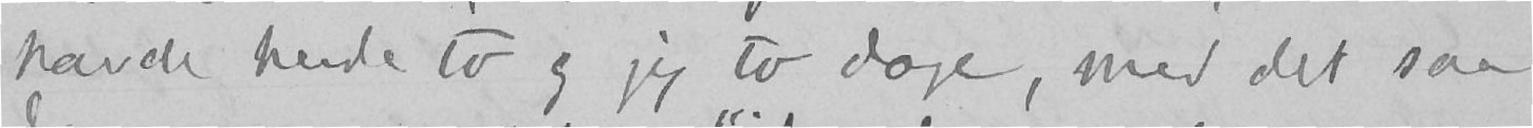havde hende to og jeg to dage, med det saa
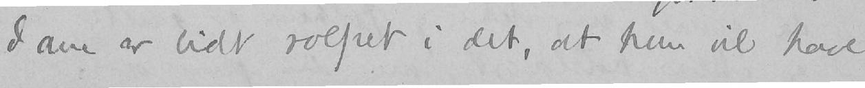Jane er lidt rolpet i det, at hun vil have
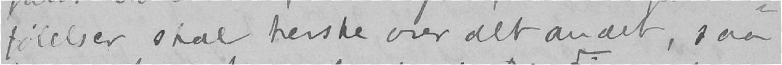følelser skal herske over det andet, saa
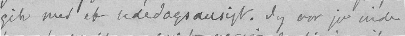gik med et bededagsansigt. Jeg var jo inde
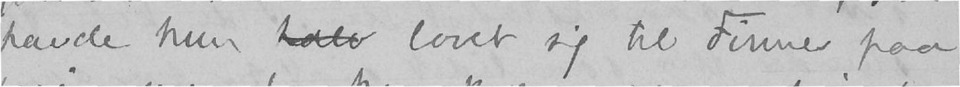have hun halv lovet sig til Finnes paa
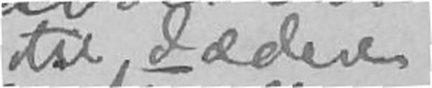til Jæderen
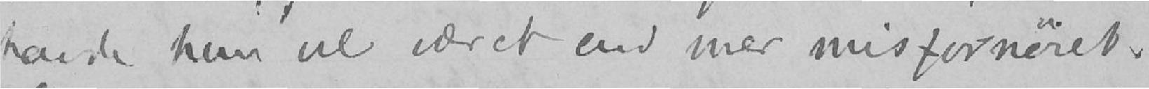havde hun vel været end mer misfornøiet.
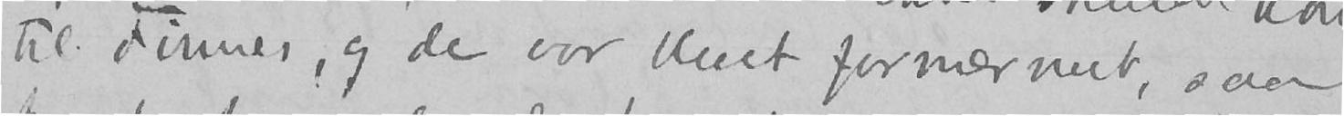til Finnes, og de var blevet fornærmet, saa
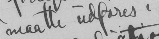maatte udfares i
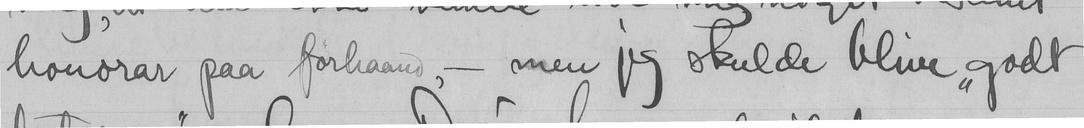honorar paa forhaand, - men jeg skulde blive "godt
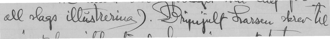all slags illustrering). Brynjulf Larsen skrev til
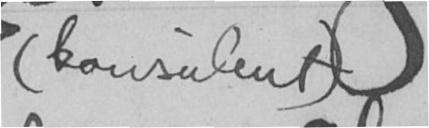(konsulent)
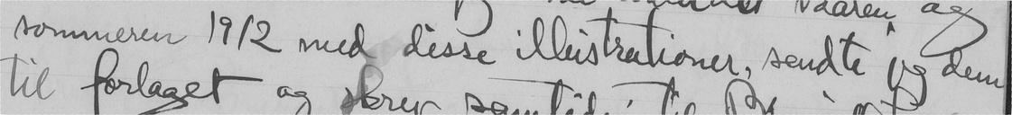sommeren 1912 med disse illustrationer, sendte jeg dem
